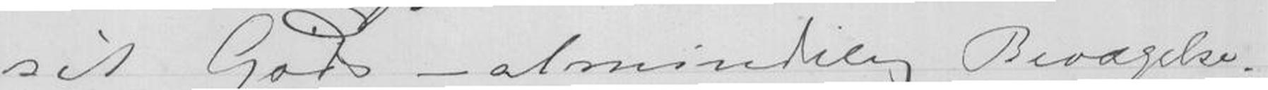sit Gods - almindelig Bevægelse.
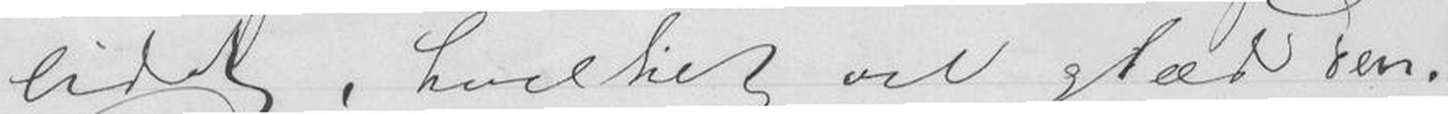lidet, hvilket vil glæde Den.
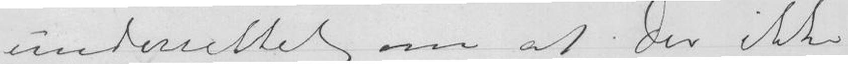underrettet om at Du ikke
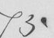75
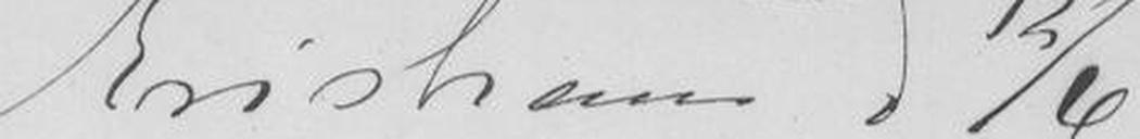Kristiania d 12/6
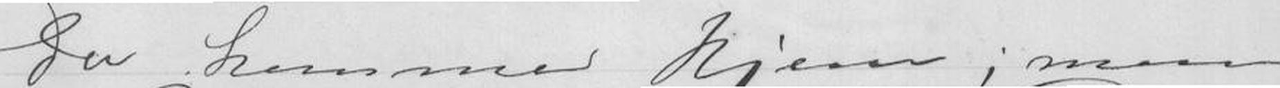Du kommer Hjem; men
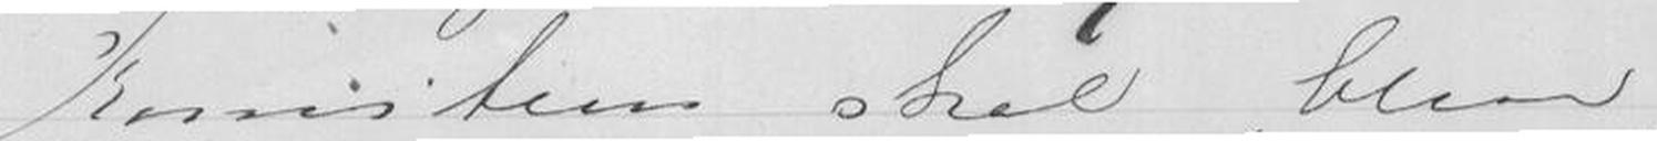Komiteen skal blive
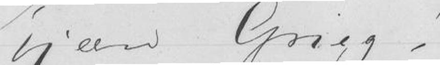Kjære Grieg!
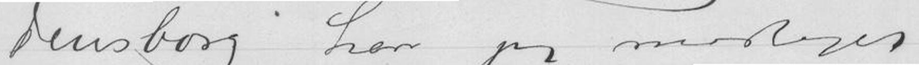densborg har jeg modtaget
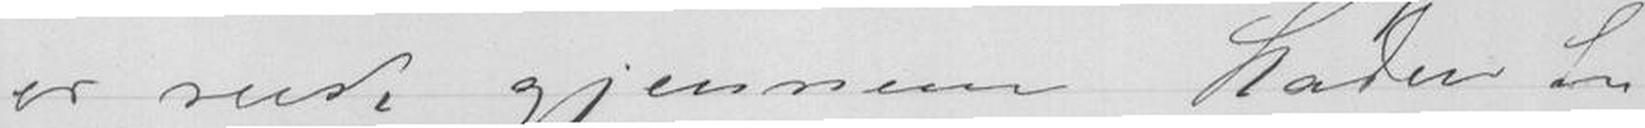er reist gjennem Staden til
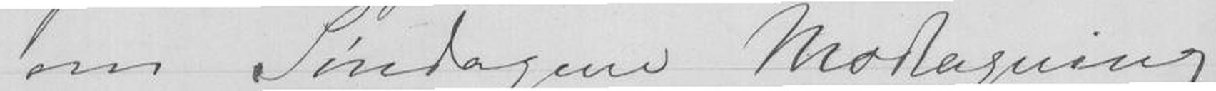om Søndagen Modtagning
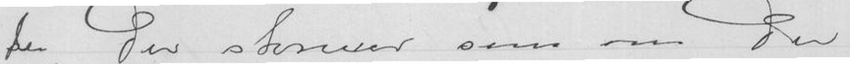da Du skriver som om Du
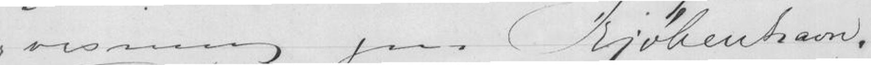visning paa Kjøbenhavn.
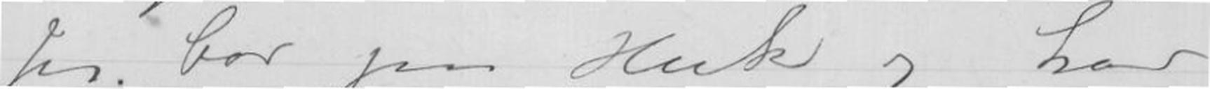Jeg bor paa Huk og har
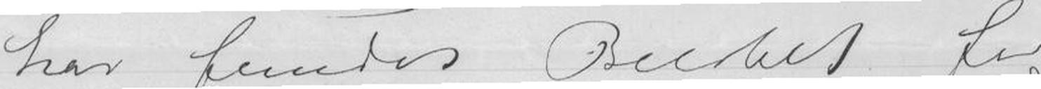har fundet Beløbet for
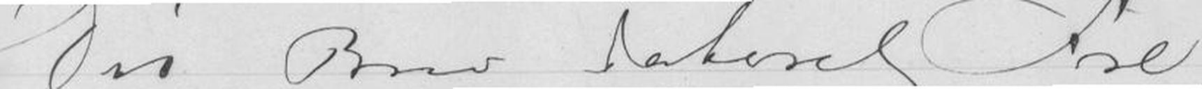Dit Brev dateret Fre-
